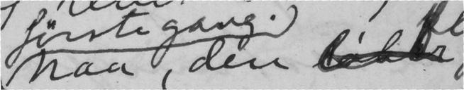naa, den
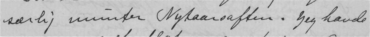særlig munter Nytaarsaften. Jeg havde
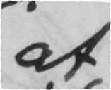at
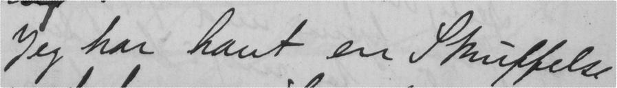Jeg har havt en Skuffelse
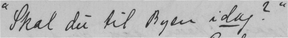"Skal du til Byen idag?"
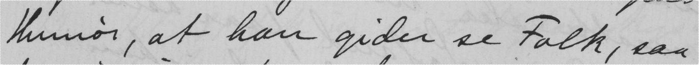Humør, at han gider se Folk, saa
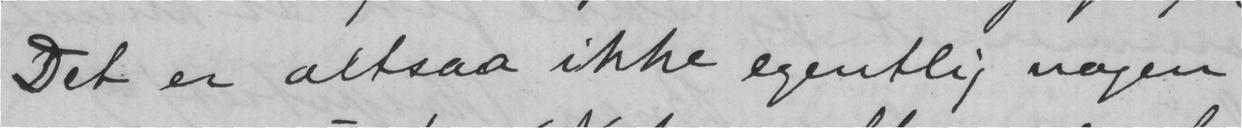Det er altsaa ikke egentlig nogen
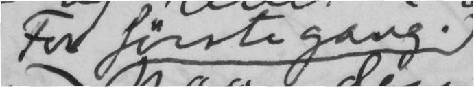For første gang.
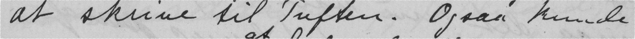at skrive til Tuften. Ogsaa kunde
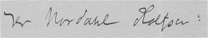Hr Nordahl Rolfsen!
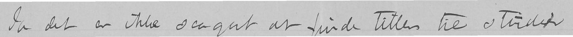Ja det er ikke saa godt at finde titler til studier
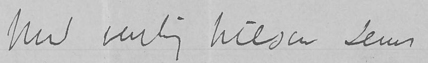Med venlig hilsen Deres
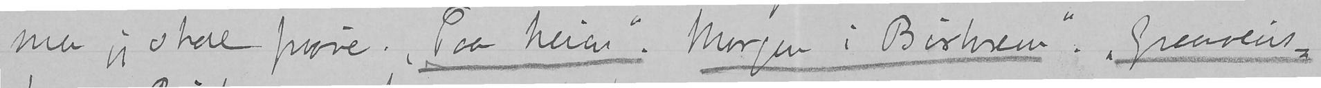men jeg skal prøve. "Paa heien". Morgen i Birkrem". Graaveirs-
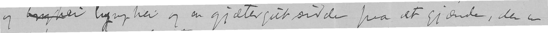og lyghei lynghei og en gjætergut sidder paa et gjærde, der er
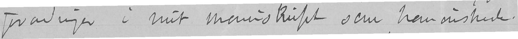forandringer i mit manuskript som han ønskede.
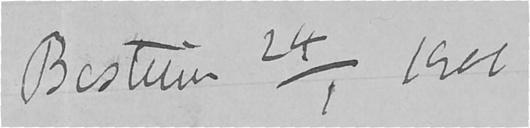Bestum 24/1 1900
